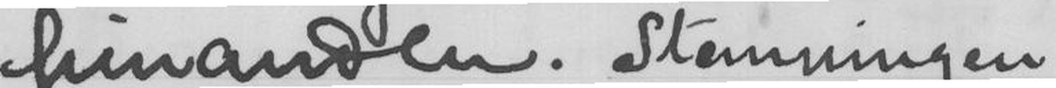hinanden. Stemningen
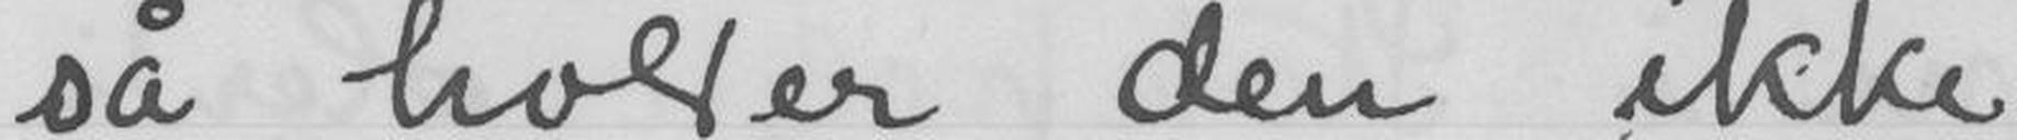så holder den ikke
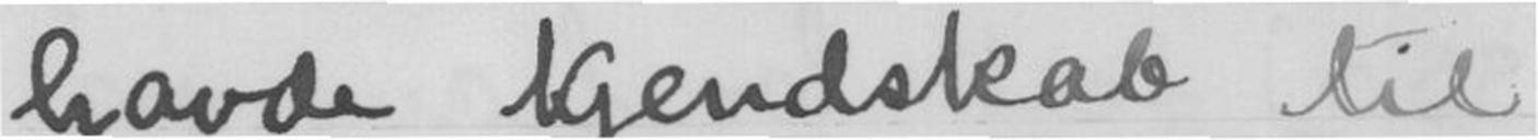havde kjendskab til
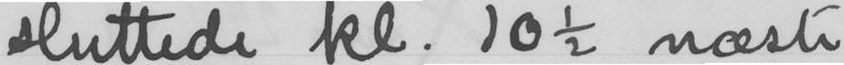sluttede kl. 10½ næste
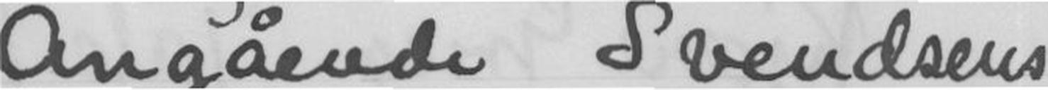Angående Svendsens
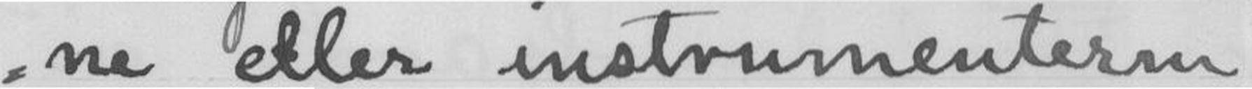ne eller instrumenterne
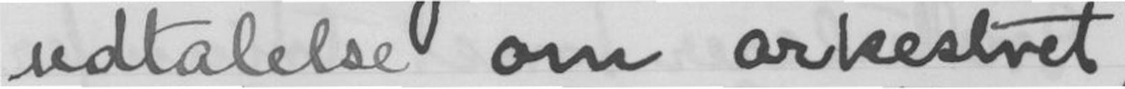udtalelse om orkestret,
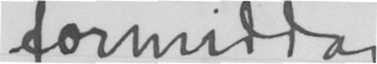formiddag. ----
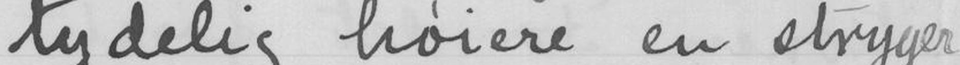tydelig høiere en stryger
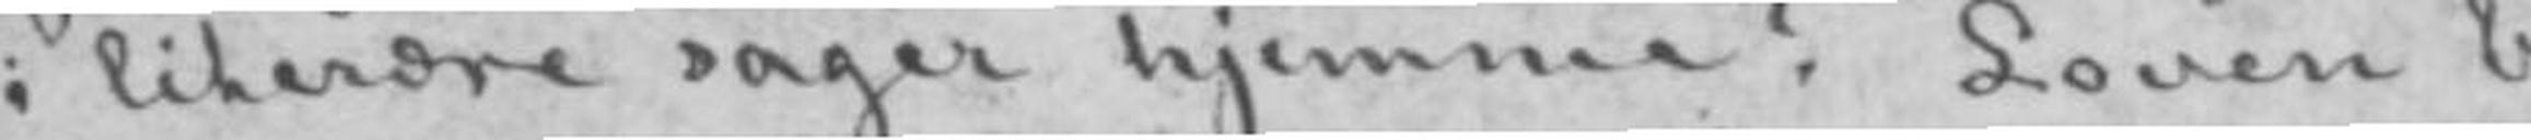i literære sager hjemme? Loven be-
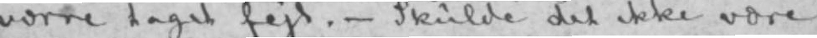værre taget fejl.- Skulde det ikke være
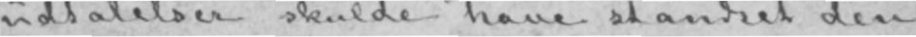udtalelser skulde have standset den
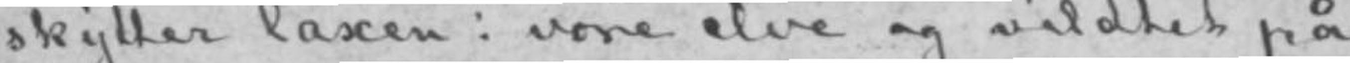skytter laxen i vore elve og vildtet på
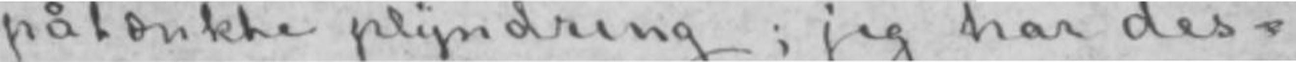påtænkte plyndring; jeg har des-
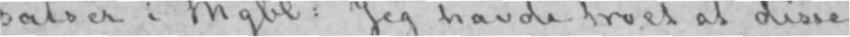satser i Mgbl: Jeg havde troet at disse
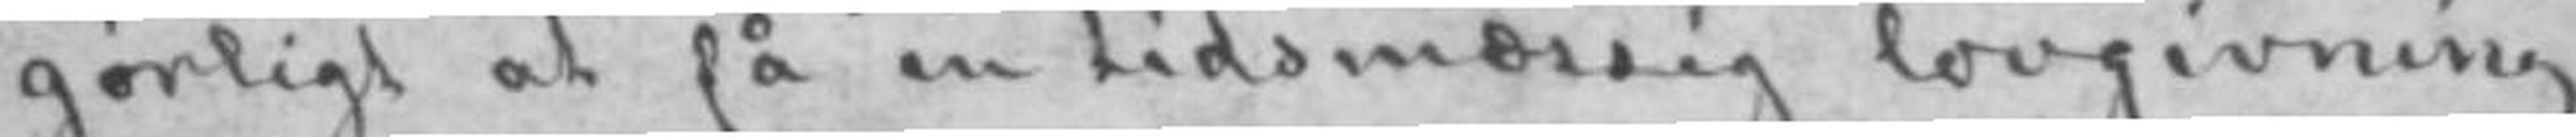gørligt at få en tidsmæssig lovgivning
